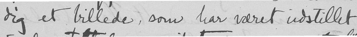dig et billede, som har været udstillet
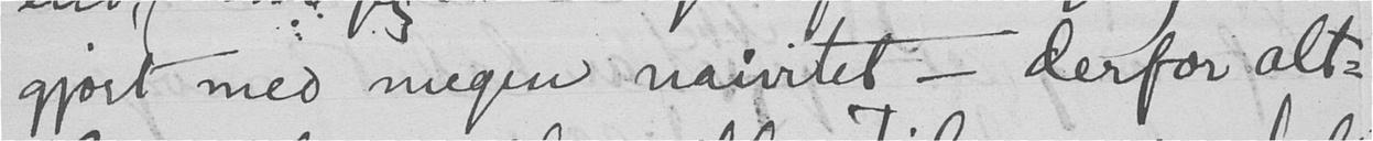gjort med megen naivitet - derfor alt-
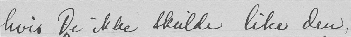hvis De ikke skulde like den,
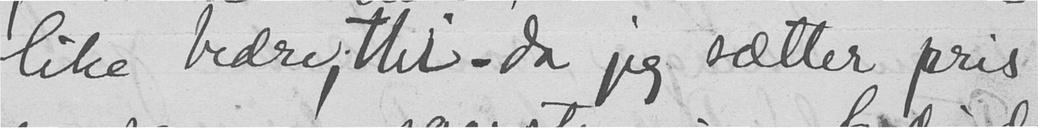like bedre; thi - da jeg sætter pris
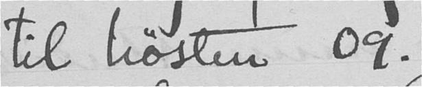til høsten 09.
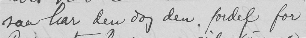saa har den dog den fordel for
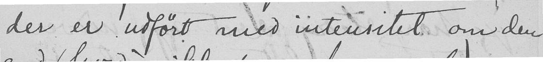der er udført med intensitet om den
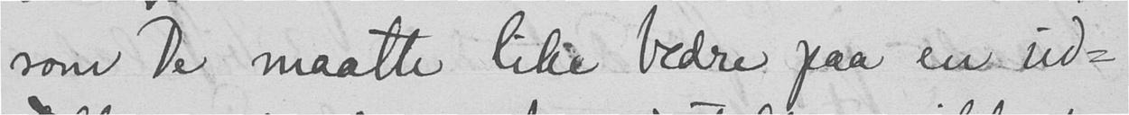som De maatte like bedre paa en ud-
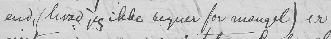end, (hvad jeg ikke regner for mangel) er
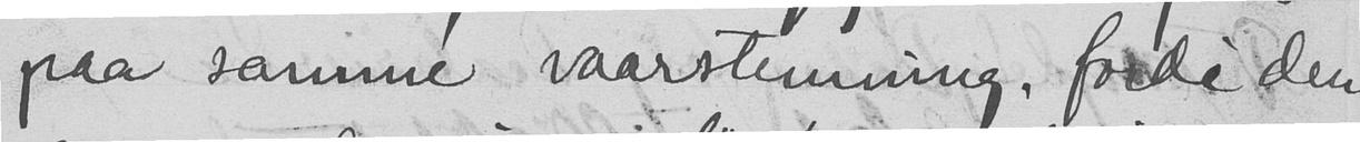paa samme vaarstemning, - fordi den
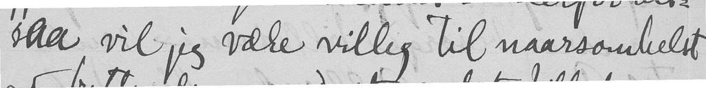saa vil jeg være villig til naarsomhelst
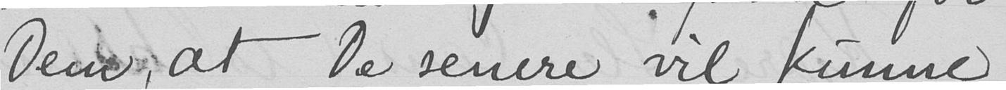Dem, at De senere vil kunne
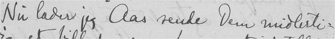Nu lader jeg Aas sende Dem midlerti-
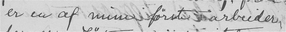er en af mine første arbeider,
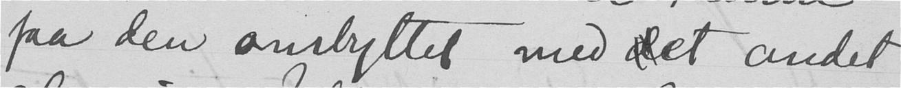faa den ombyttet med et andet
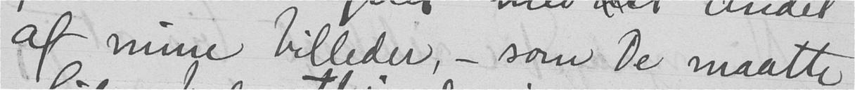af mine billeder, - som De maatte
